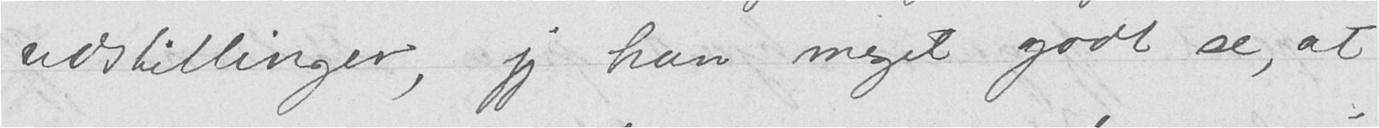udstillinger, jeg kan meget godt se, at
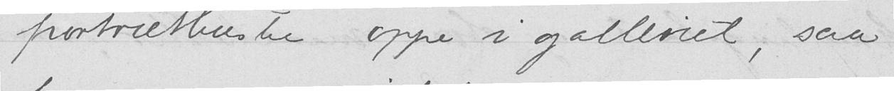portrætbuste oppe i galleriet, saa
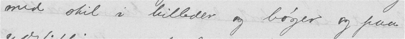med stil i billeder og bøger og paa
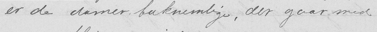er de damer taknemlige, der gaar med
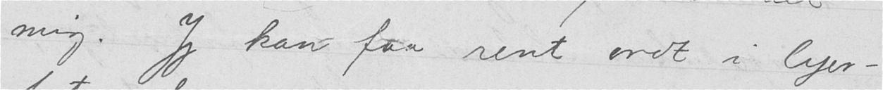mig. Jeg kan faa rent ondt i brys-
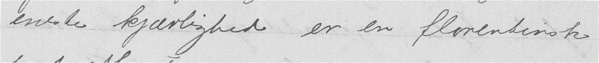eneste kjærlighed er en florentinsk
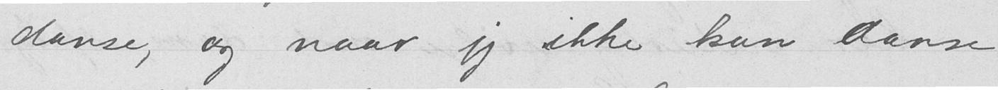danse, og naar jeg ikke kan danse
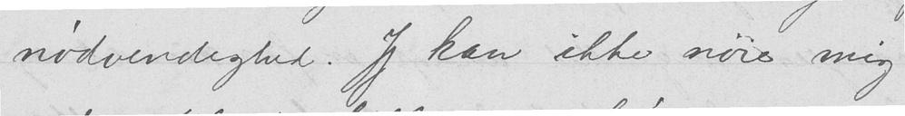nødvendighed. Jeg kan ikke nøie mig
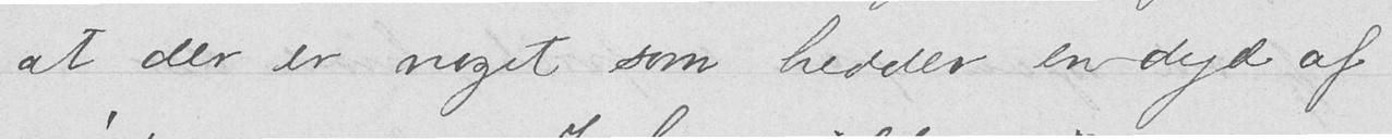at der er noget som hedder en dyd af
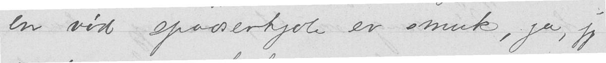en rød spadserkjole er smuk, ja, jeg
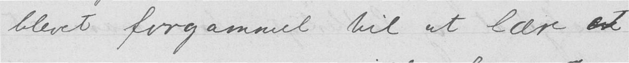blevet forgammel til at lære at
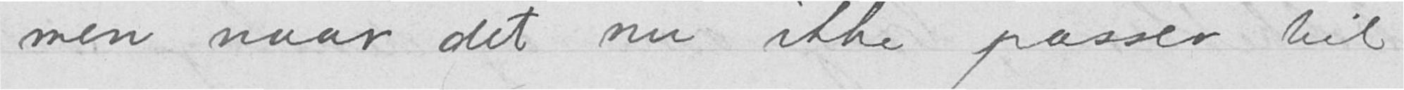men naar det nu ikke passer til
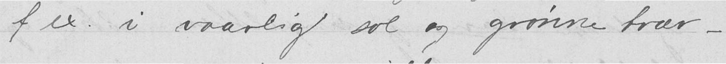f ex. i vaarlig sol og grønne trær -
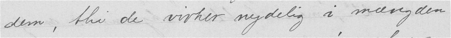dem, thi de virker nydelig i mængden
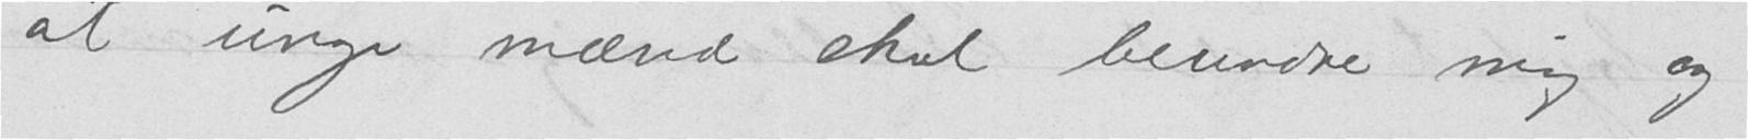at unge mænd skal beundre mig og
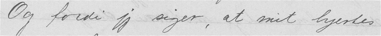Og fordi jeg siger, at mit hjertes
